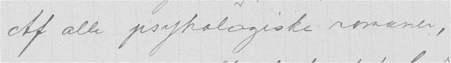Af alle psykologiske romaner,
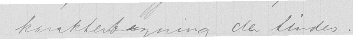karaktertegning, der findes.
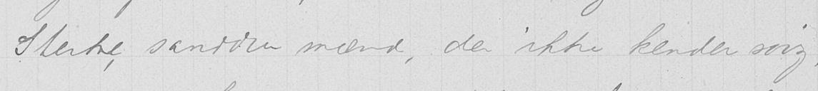Sterke, sanddru mænd, der ikke kender svig,
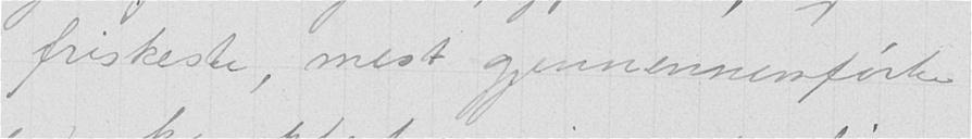friskeste, mest gjennemførte
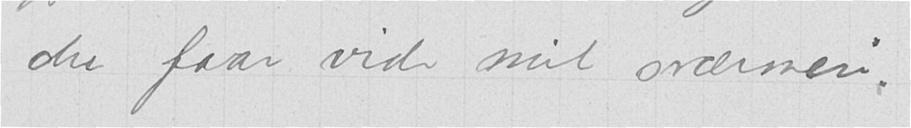du faar vide mit sværmeri.
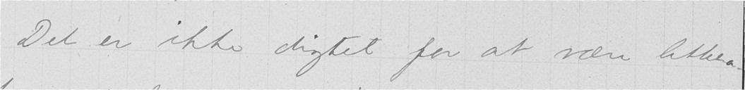Det er ikke digtet for at være litte-
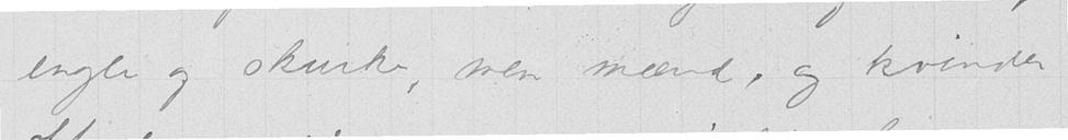engle og skurke, men mænd, og kvinder.
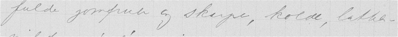fulde jomfruer og skarpe, kolde, latter-
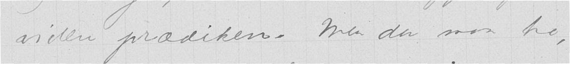videre prædiken. Men du maa tro,
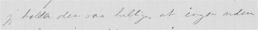jeg holder dem saa hellige, at ingen uden
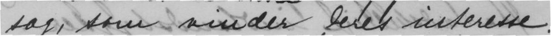sag, som vinder Deres interesse.
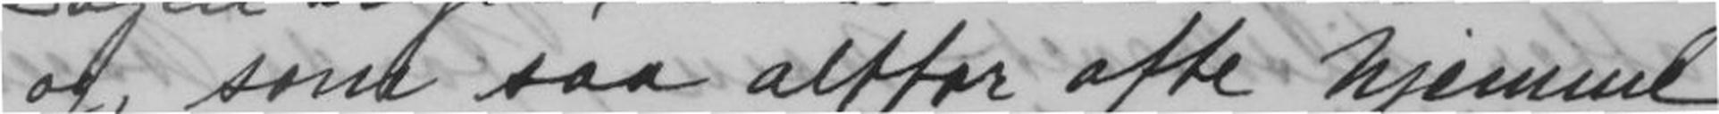og, som saa altfor ofte hjemme,
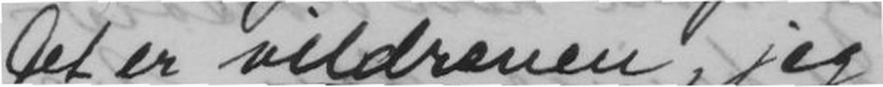Det er vildrenen, jeg
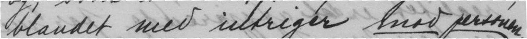blandet med intriger mot personen.
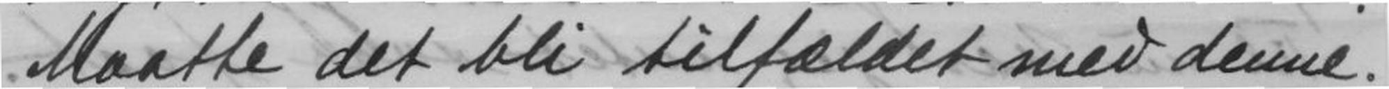Maatte det bli tilfældet med denne.
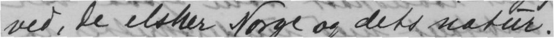ved, De elsker Norge og dets natur.
